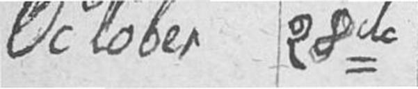October 28de
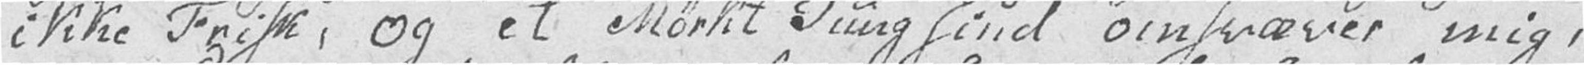ikke Frisk, og et Mørkt Tungsind omsvæver mig,
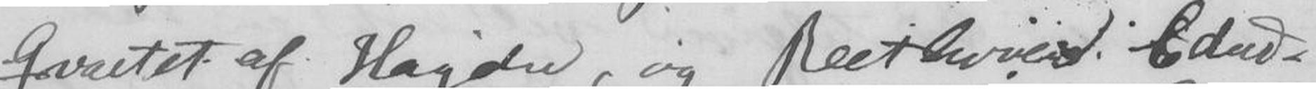Qvartet af Haydn, og Beethovens Cdur-
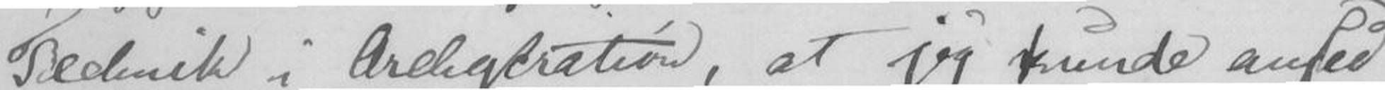Technik i Orchestration, at jeg kunde ansee
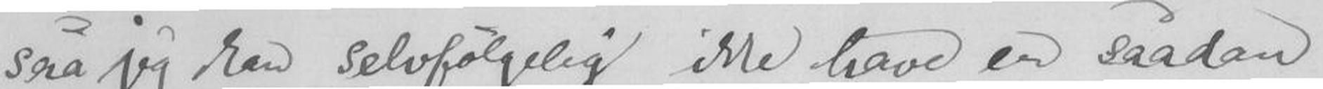saa jeg kan selvfølgelig ikke have en saadan
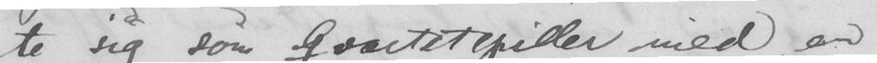te sig som Qvartetspiler med en
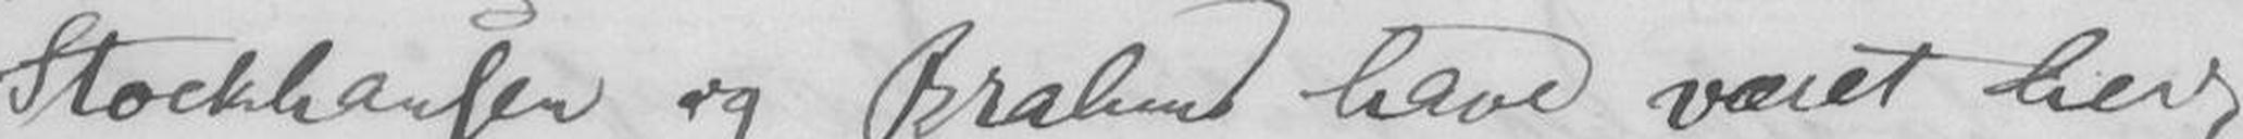Stockhansen og Brahms have været her
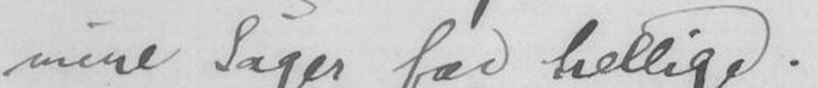mine Sager for hellige.
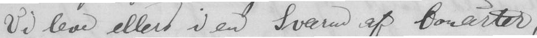Vi leve ellers i en Sværm af Concerter,
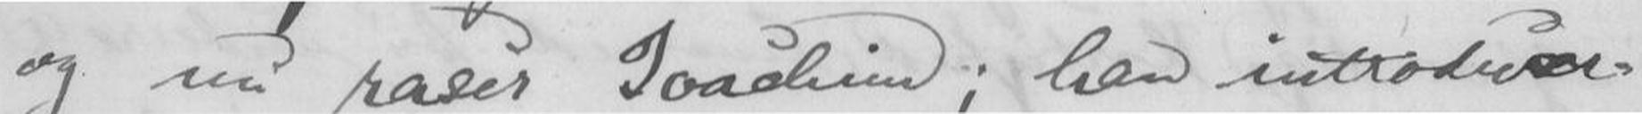og nu raser Joachim; han introduser-
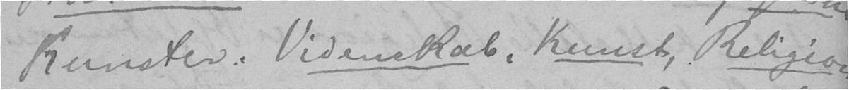Kunster. Videnskab, Kunst, Religion
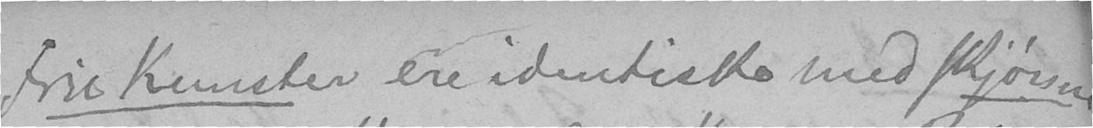Frie kunster ere identiske med skjønne
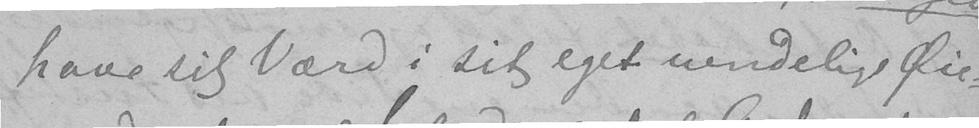have sit Værd i sit eget uendelige Øie-
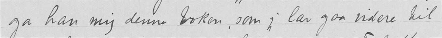ga han mig denne boken, som jeg lar gaa videre til
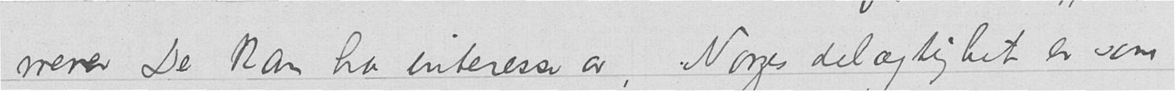mener De kan ha interesse av, Norges delagtighet er som
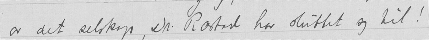av det selskap, Dr. Rostad har sluttet sig til!
